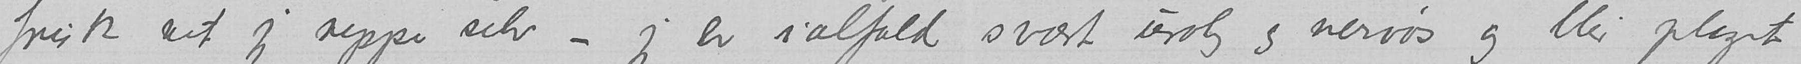frisk vet jeg neppe selv – jeg er ialfald svært urolig og nervøs og blir plaget
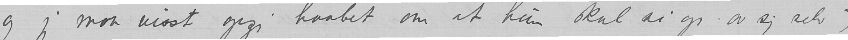og jeg maa visst opgi haabet om at hun skal si op av sig selv),
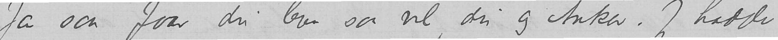Ja saa faar du leve saa vel, du og Anker. Jeg hadde
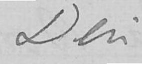Din
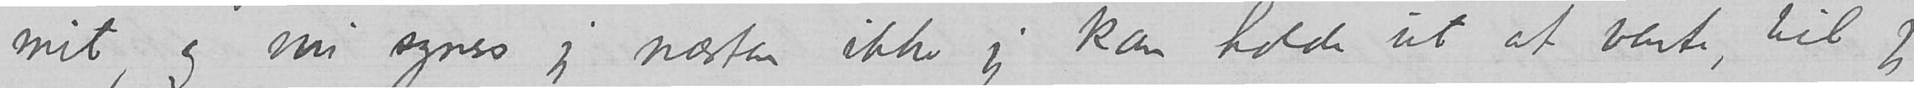mit, og nu synes jeg næsten ikke jeg kan holde ut at vente, til jeg
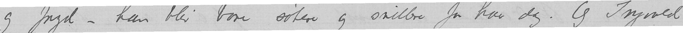og fryd – han blir bare søtere og snillere for hver dag. Og Ingvald
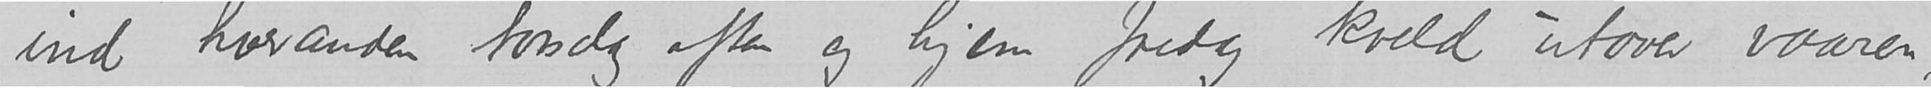ind hveranden torsdag aften og hjem fredag kveld utover vaaren,
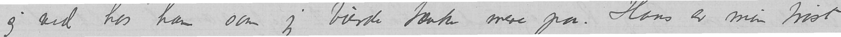sig ved hos ham som jeg burde tænke mere paa. Hans er min trøst
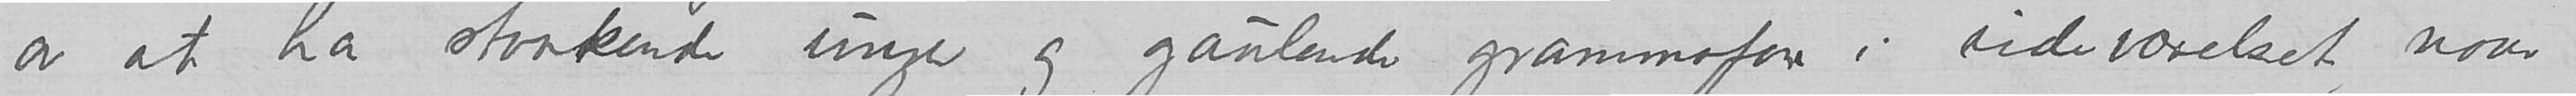av at ha staakende unger og gaulende grammofoner i sideværelset, naar jeg
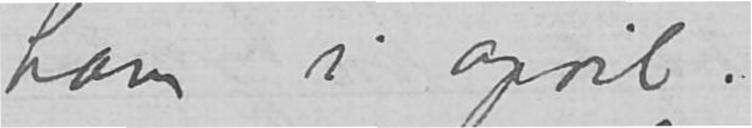ham i april.
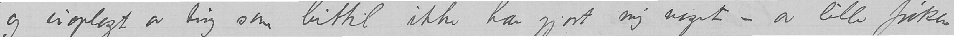og uoplagt av ting som hittil ikke har gjort mig noget – av lille frøken
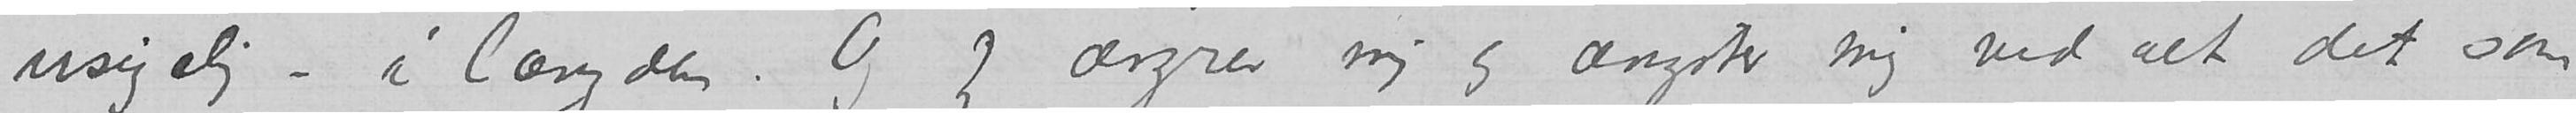usigelig – i længden. Og jeg ærgrer mig og ængster mig med alt det som
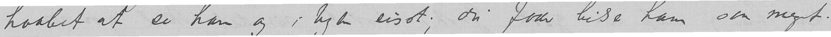haabet at se ham og i byen sisst; du faar hilse ham saa meget.
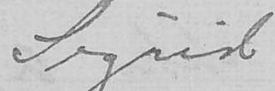Sigrid.
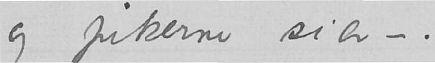og pikerne sier –.
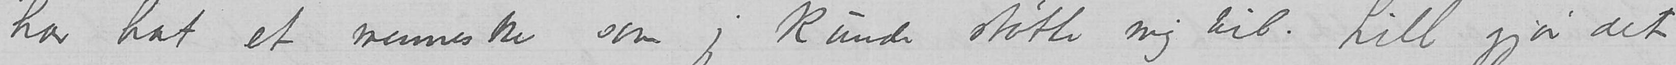har hat et menneske som jeg kunde støtte mig til. Litt gjør det
